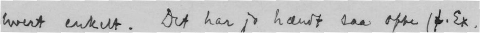hvert enkelt. Det har jo hændt saa ofte (f. Ex.
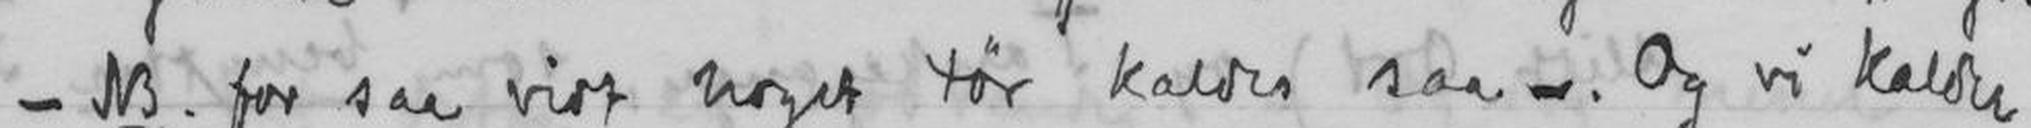- N. B. for saa vidt noget tør kaldes saa. - Og vi kalde
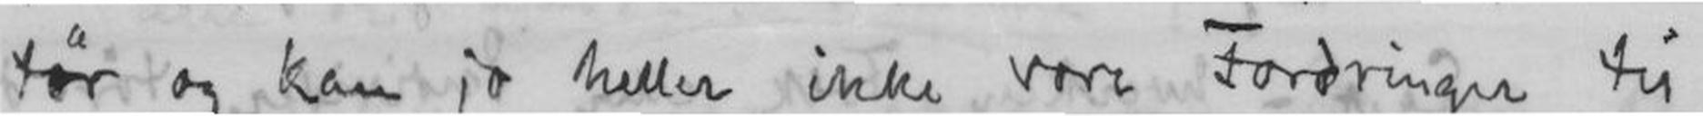tør og kan jo heller ikke vore Fordringer til
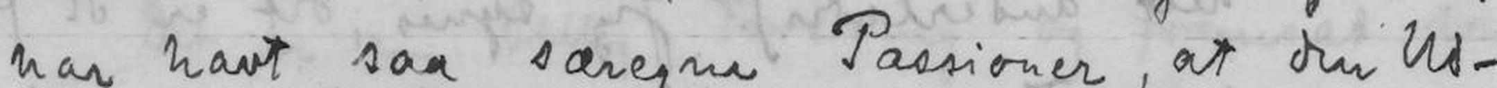har havt saa særegne Passioner, at den Ud-
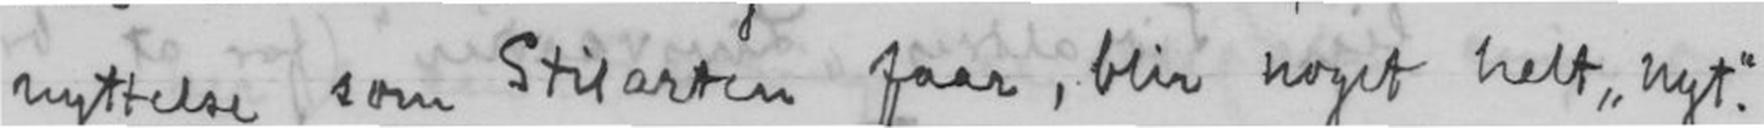nyttelse som Stilarten faar, blir noget helt nyt.
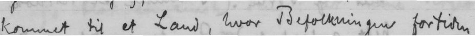kommet til et Land, hvor Befolkningen fortiden
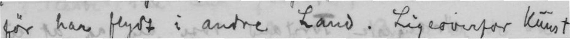før har flydt i andre Land. Ligeoverfor Kunst
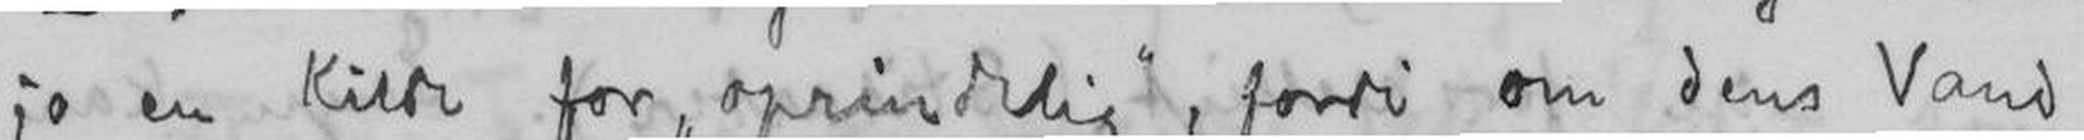jo en kilde for oprindlig, fordi om dens Vand
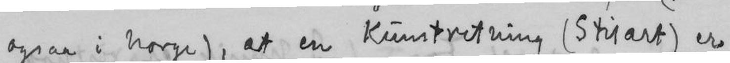ogsaa i Norge), at en Kunstretning (Stilart) er
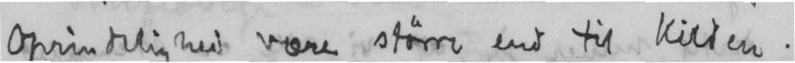Oprindlighed være større end til Kilden.
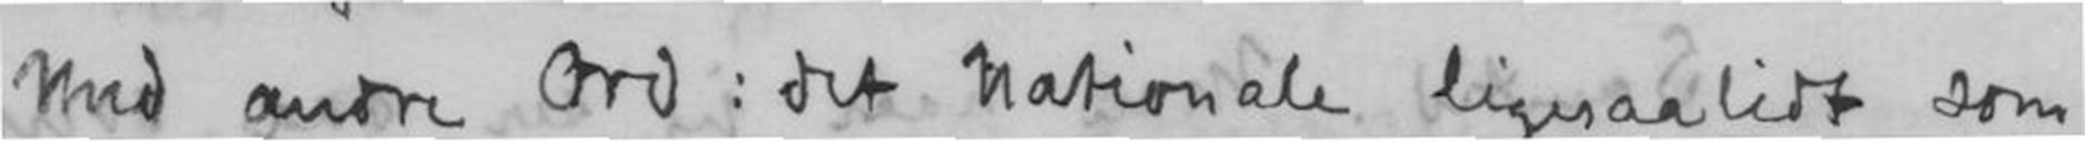Med andre Ord: det nationale ligesaalidt som
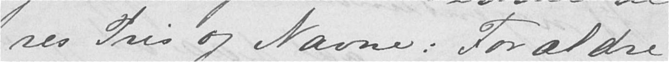res Pris og Navne: Forældre-
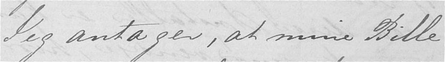Jeg antager, at mine Bille-
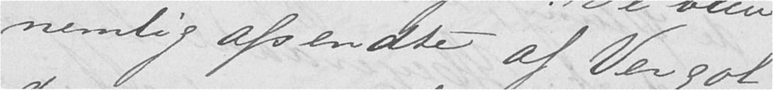nemlig afsendte af Vergol-
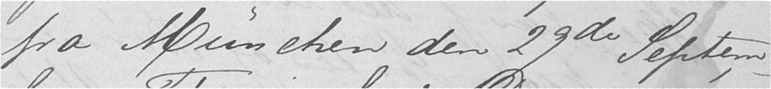fra München den 29de Septem-
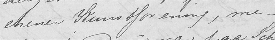chener Kunstforening, me-
